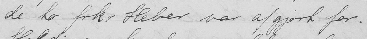de to frk.r Heber var afgjort for.
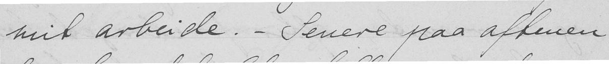mit arbeide. - Senere paa aftenen
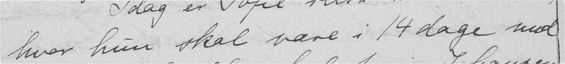hvor hun skal være i 14 dage med
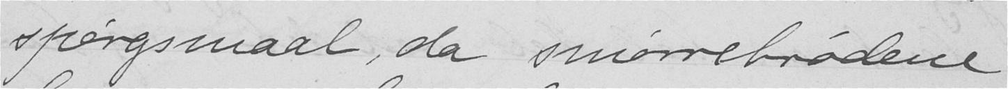spørgsmaal, da smørrebrødene
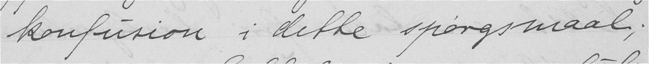konfusion i dette spørgsmaal;
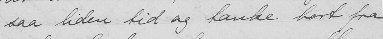saa liden tid og tanke bort fra
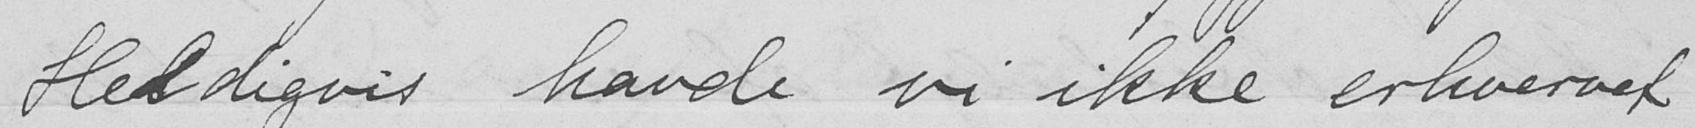Heldigvis havde vi ikke erhvervet
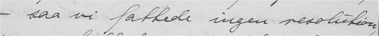- saa vi fattede ingen resolution,
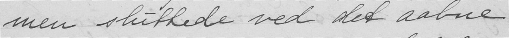men sluttede ved det aabne
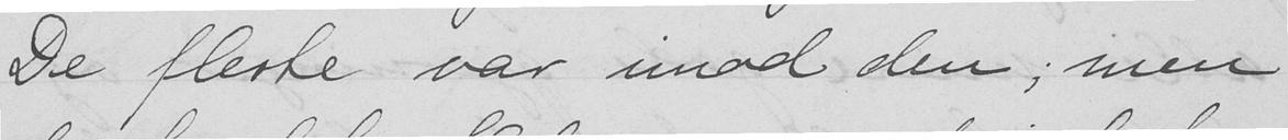De fleste var imod den; men
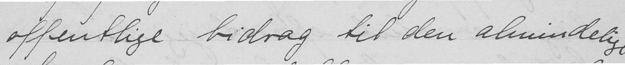offentlige bidrag til den almindelige
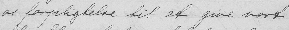os forpligtelse til at give vort
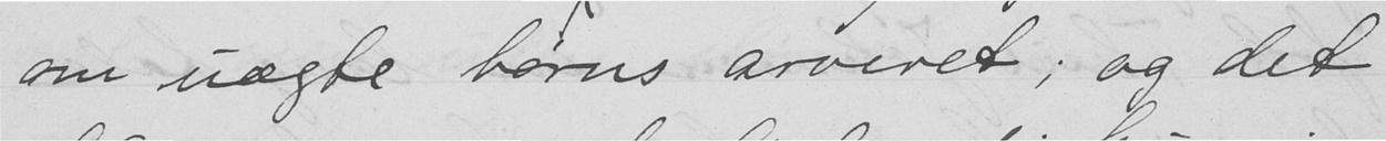om uægte børns arveret; og det
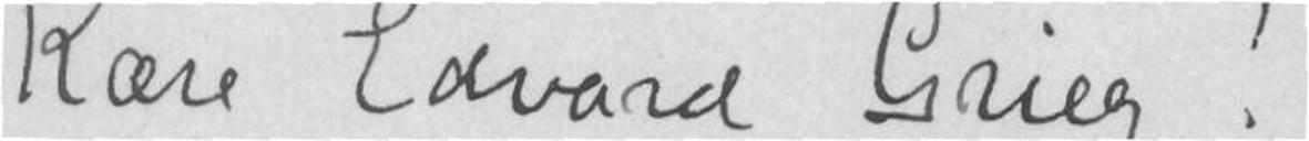Kære Edvard Grieg!
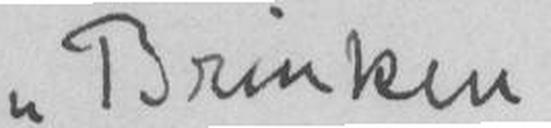"Brinken"
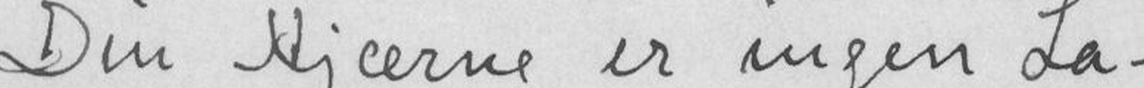Din Hjærne er ingen La-
